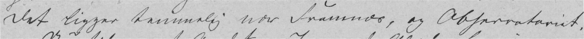Det ligger temmelig nær Framnæs, og Observatoriet.
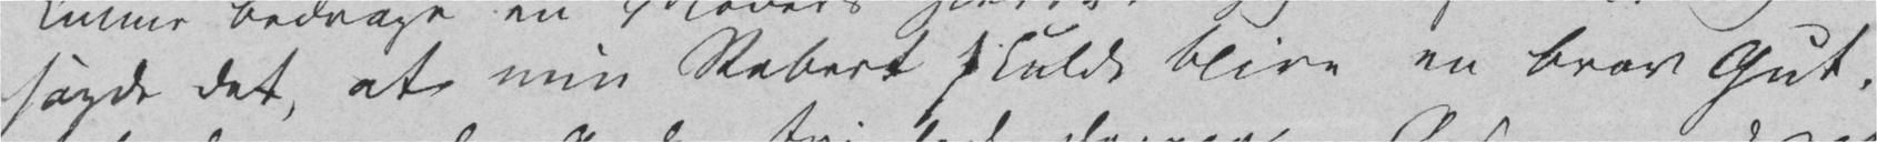sagde det, at min Robert skulde blive en brav Gut,
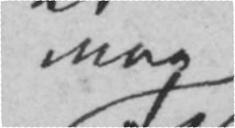maa
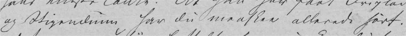og Stipendium har Du maaske allerede hørt.
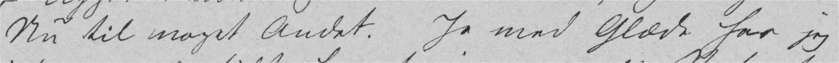Nu til noget Andet. Ja med Glæde har jeg
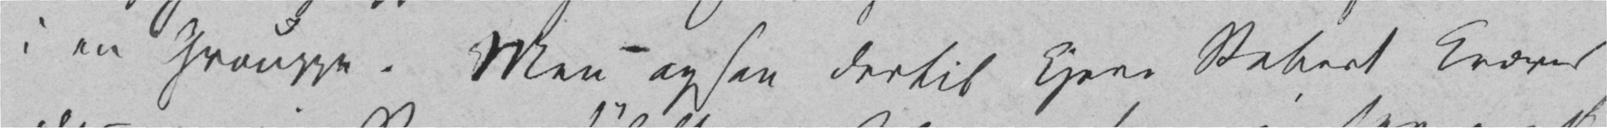i en Grouppe. Men ogsaa dertil kjere Robert kræves
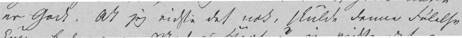er Godt. Ak jeg vidste det nok, skulde denne Følelse
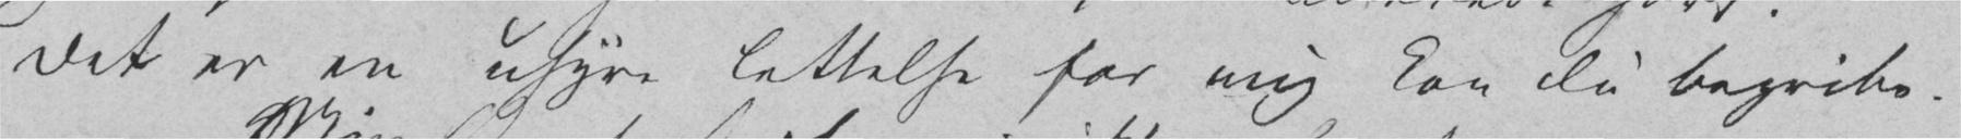Det er en uhyre Lettelse for mig kan Du begribe.
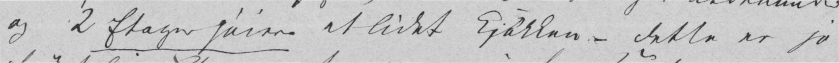og 2 Etager høiere et lidet Kjøkken – dette er jo
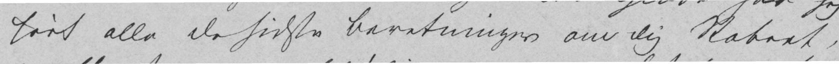hørt alle de sidste Beretninger om Dig Robert,
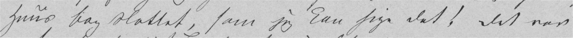Huus bag Slottet, som jeg kan sige det! Det var
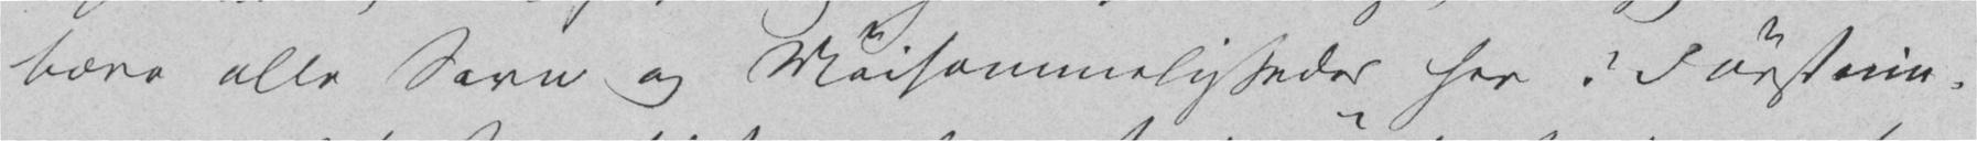bære alle Savn og Møisommeligheder her i Førstnin-
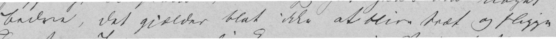bedre, det gjælder blot ikke at blive træt og slippe
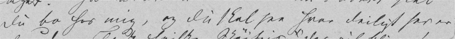Du bo hos mig, og Du skal see hvor deiligt her er
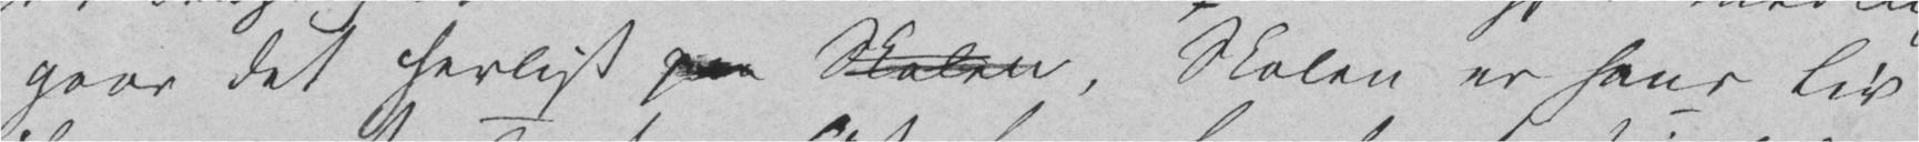gaar det herligt paa Skolen, Skolen er hans Liv
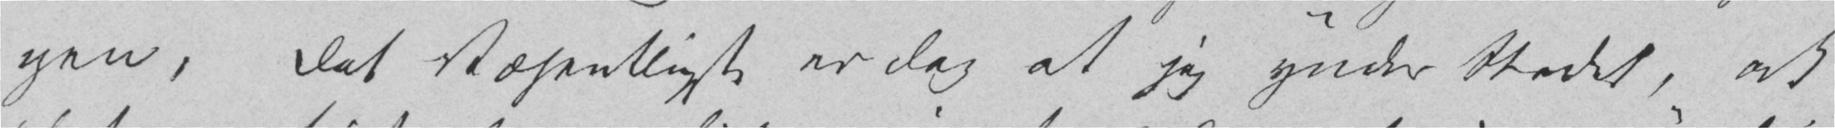gen, det Væsentligste er dog at jeg ynder Stedet, ak
